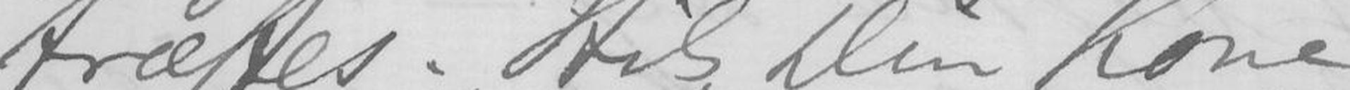træffes. Hils Din Kone og
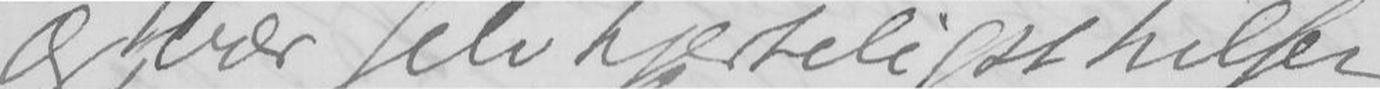vær selv hjerteligst hilset
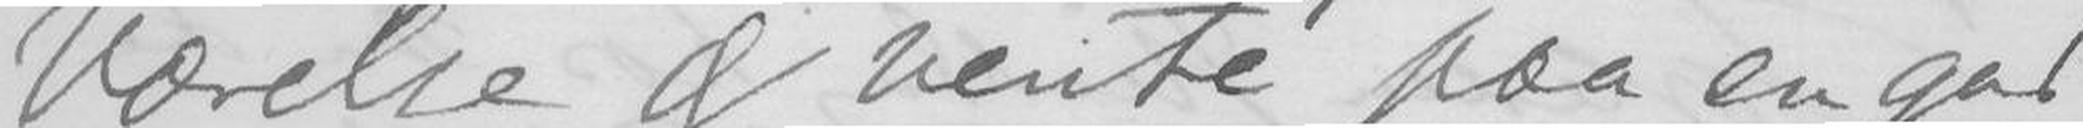Værelse og vente paa en god
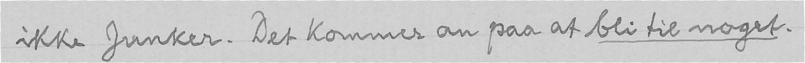ikke Junker. Det kommer an paa at bli til noget.
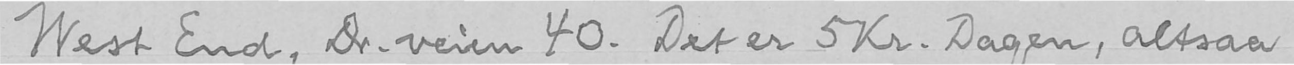West End, Dr.veien 40. Det er 5 Kr. Dagen, altsaa
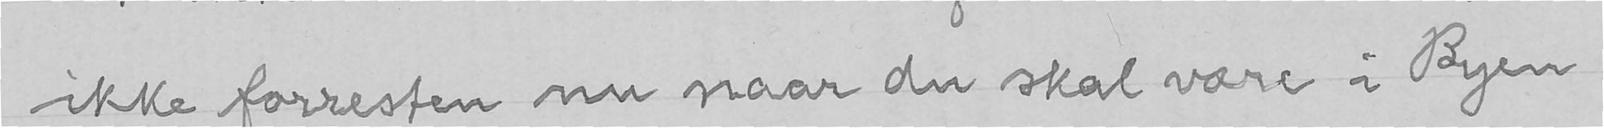ikke forresten nu naar du skal være i Byen
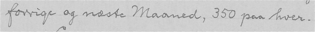forrige og næste Maaned, 350 paa hver.
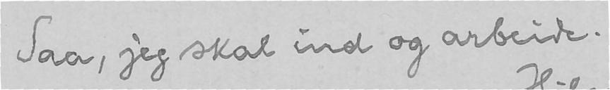Saa, jeg skal ind og arbeide.
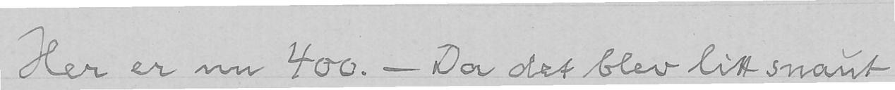Her er nu 400. - Da det blev litt snaut
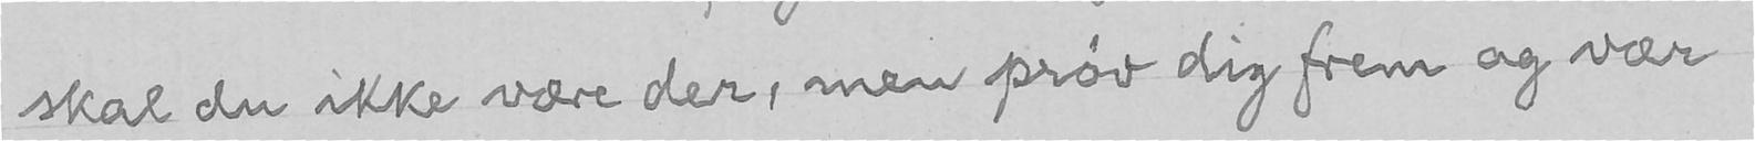skal du ikke være der, men prøv dig frem og vær
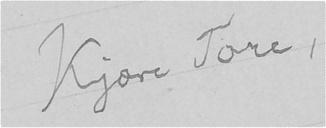Kjære Tore,
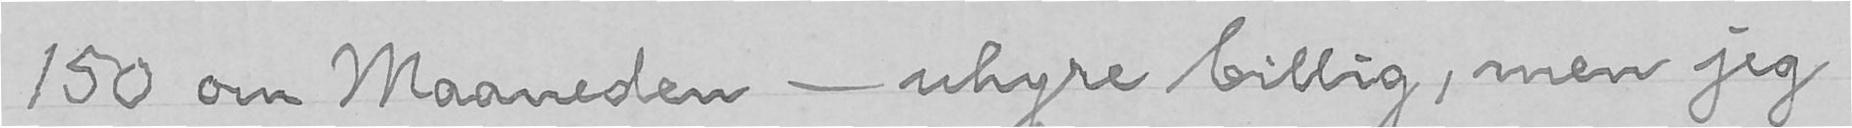150 om Maaneden - uhyre billig, men jeg
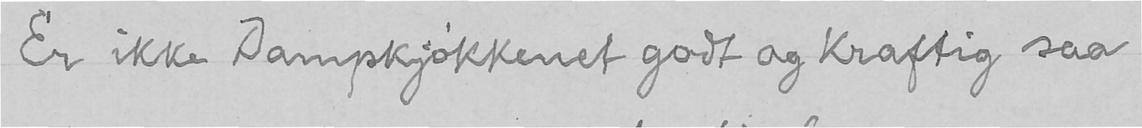Er ikke Dampkjøkkenet godt og kraftig saa
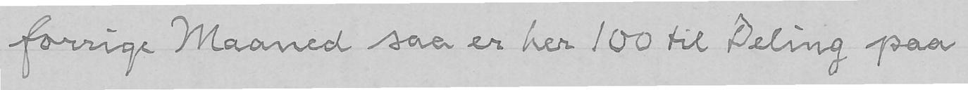forrige Maaned saa er her 100 til Deling paa
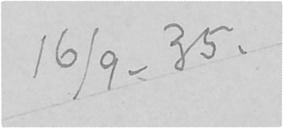16/9. 35.
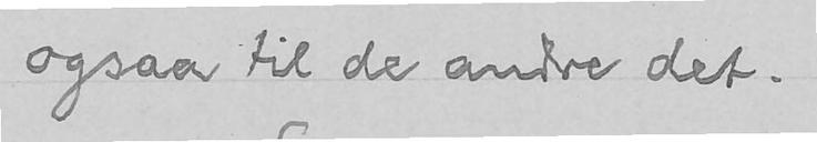ogsaa til de andre det.
